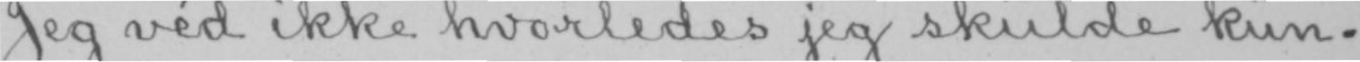Jeg véd ikke hvorledes jeg skulde kun-
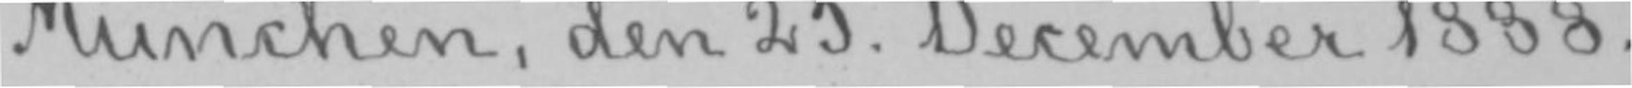München, den 25. December 1888.
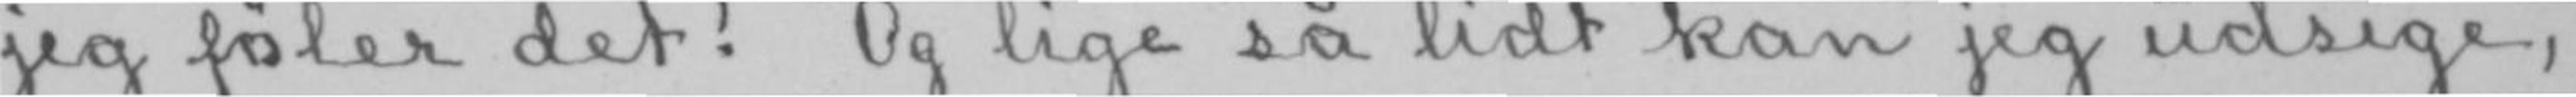jeg føler det! Og lige så lidt kan jeg udsige,
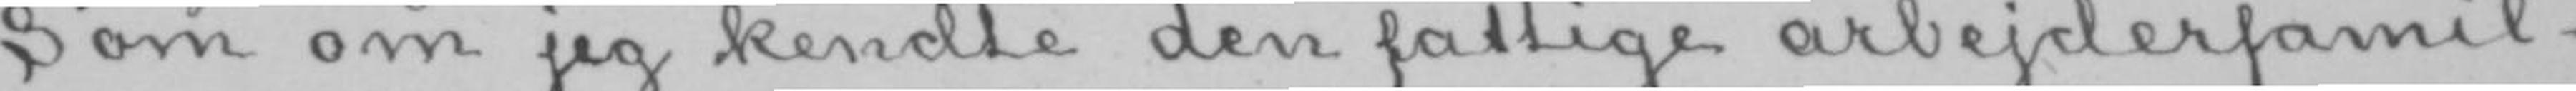Som om jeg kendte den fattige arbejderfamil-
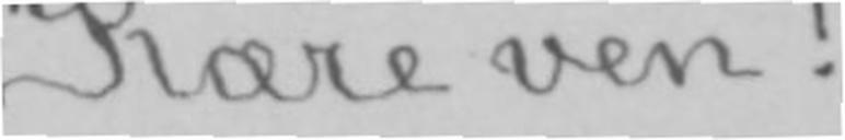Kære ven!
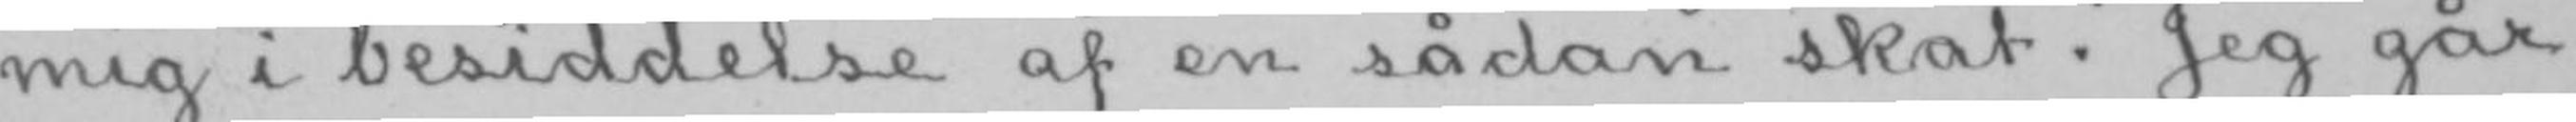mig i besiddelse af en sådan skat. Jeg går
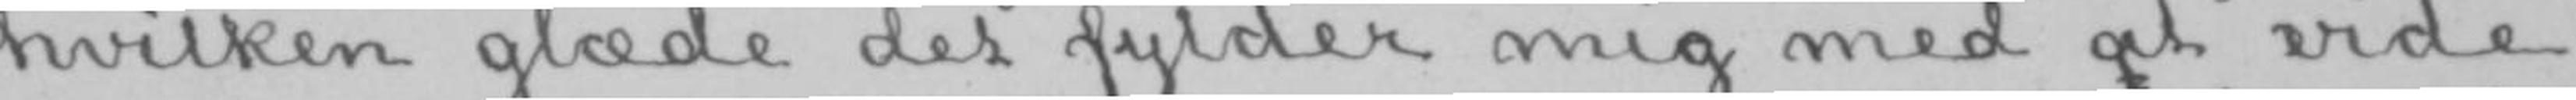hvilken glæde det fylder mig med at vide
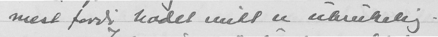mest fordi hodet mitt er ubrukelig.
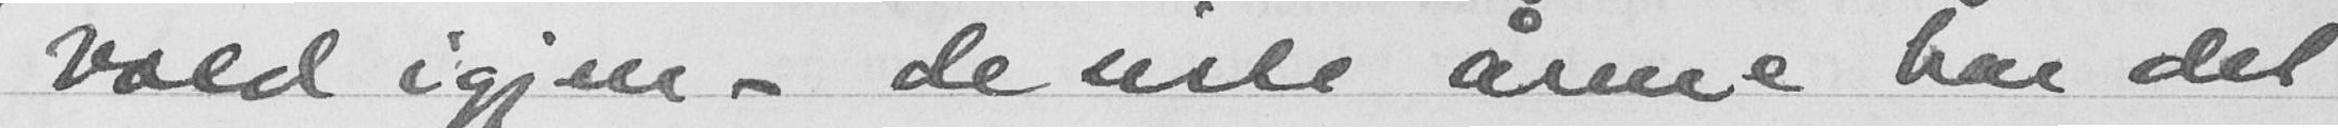vold igjen - de siste årene har det
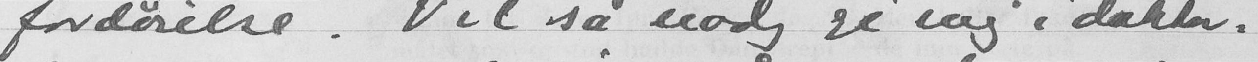fordøielse. Vil sa nødig gi mig i doktor-
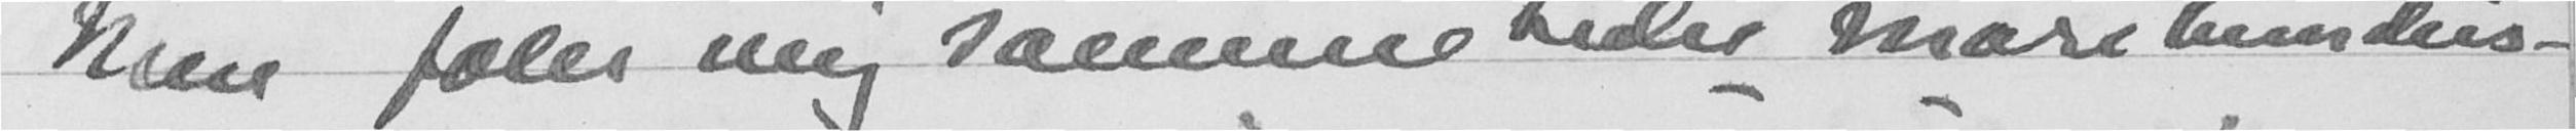Men føler mig sommetider marihunders-
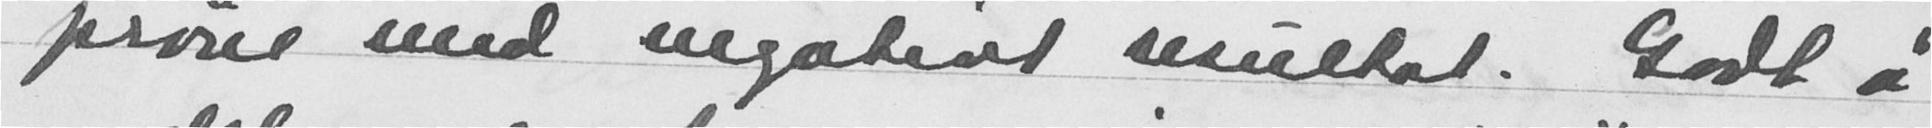prøve med negativt resultat. Godt å
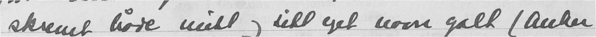skrevet både mitt og sitt eget navn galt (Anker
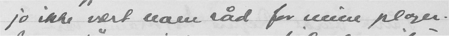jo ikke vært noen råd for mine plager.
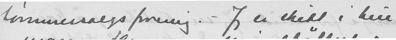tømmersalgsforening. - Jeg er skitt i hue
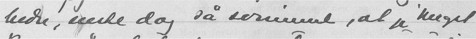bedre, neste dag så svimmel, at jeg knapt
